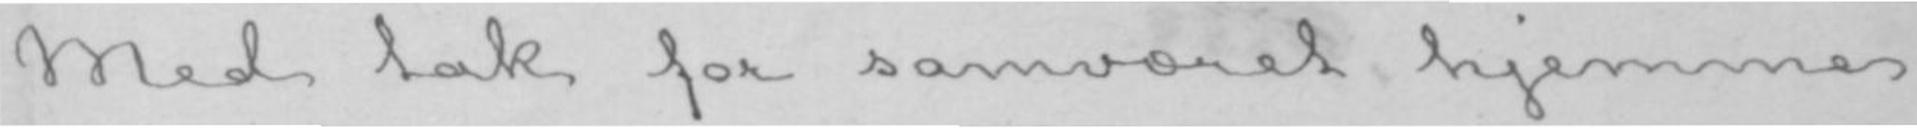Med tak for samværet hjemme
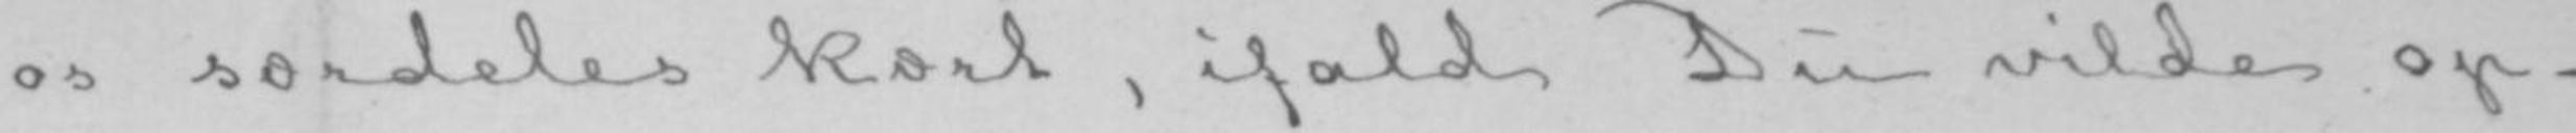os særdeles kært, ifald Du vilde op-
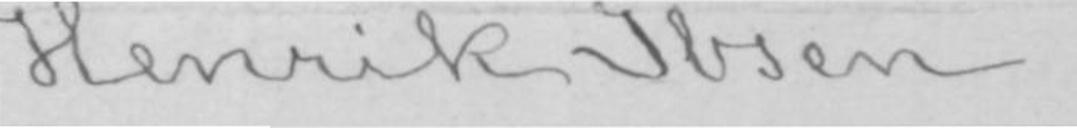Henrik Ibsen.
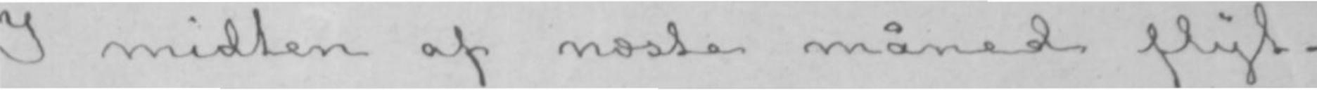I midten af næste måned flyt-
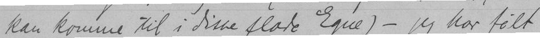kan komme til i disse flade Egne) - jeg har følt
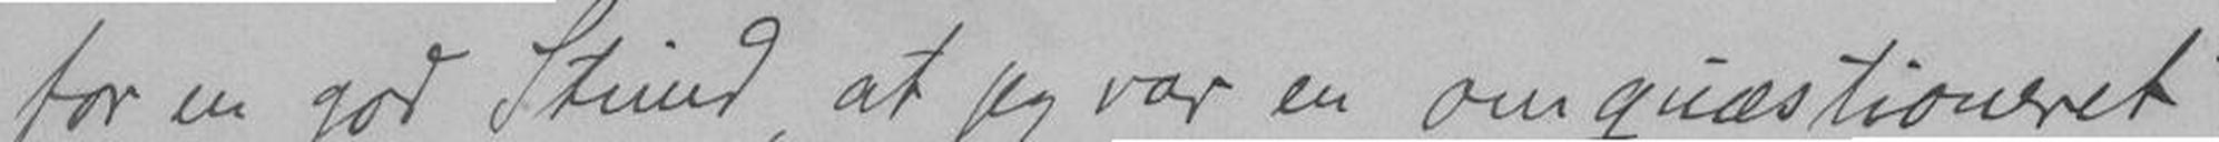for en god Stund, at jeg var en "Omquæstioneret"
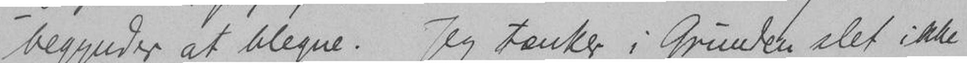begynder at blegne. Jeg tænker i Grunden slet ikke
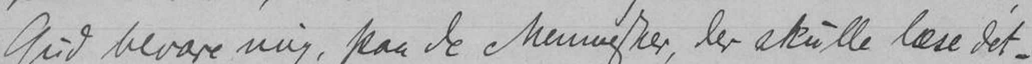Gud bevare nig, paa de Mennesker, der skulle læse det,
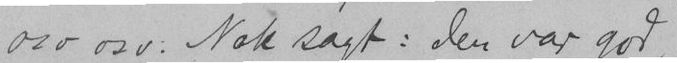osv osv. Nok sagt: den var god,
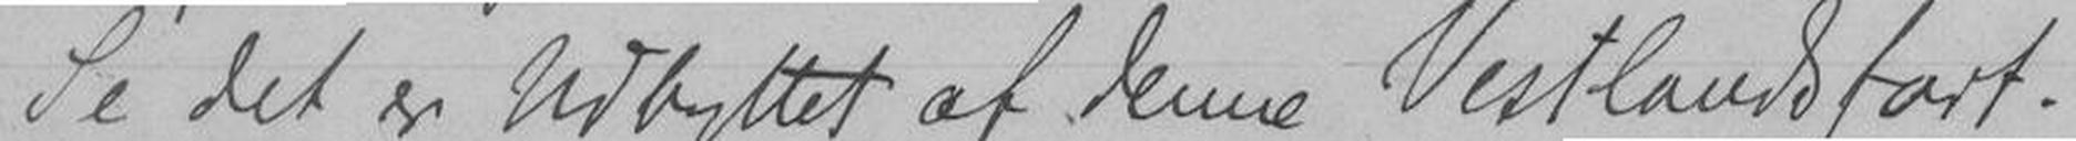Se det er Udbyttet af denne Vestlandsfart.
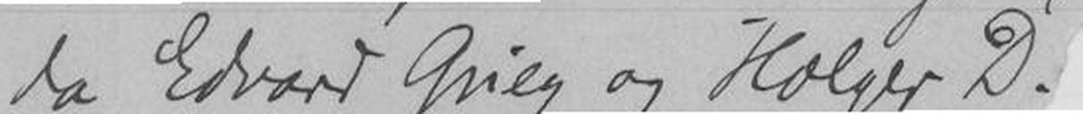da Edvard Grieg og Holger D.
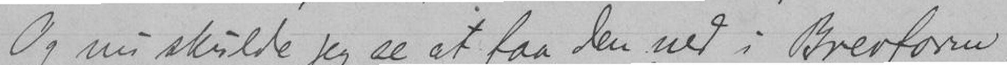Og nu skulde jeg se at faa den ned i Brevform,
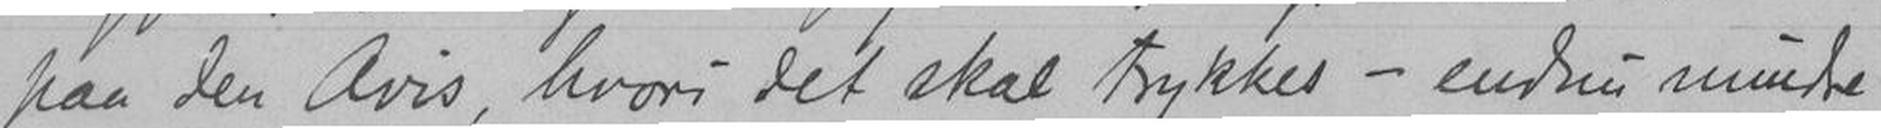paa den Avis, hvori det skal trykkes - endnu mindre
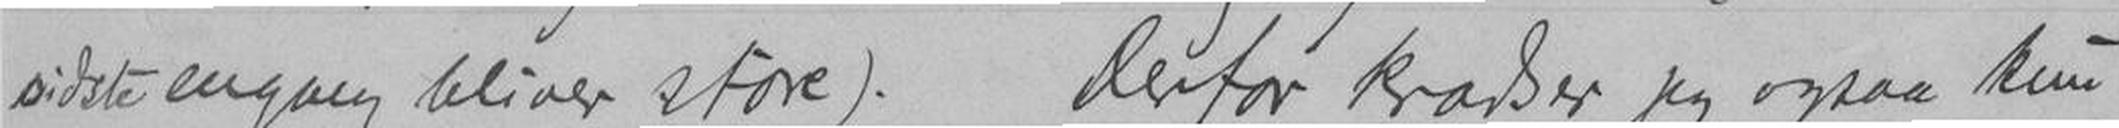sidste engang bliver store). Derfor kradser jeg ogsaa kun
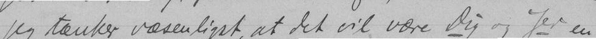jeg tænker væsentligst, at det vil Dig og Jer en
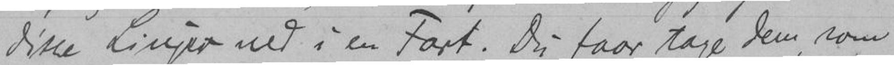disse Linjer ned i en Fart. Du faar tage dem som
